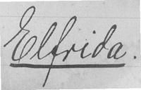Elfrida.
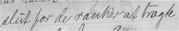slut for de ranker at tragte
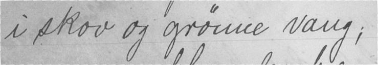i skov og grønne vang;
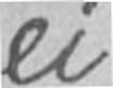ei
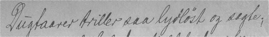Dugtaarer triller saa lydløst og sagte;
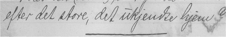efter det store, det ukjendte hjem?
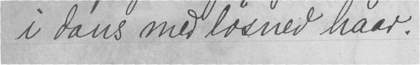i dans med løsned haar.
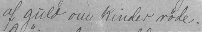af guld om kinder røde.
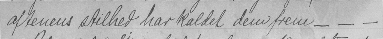aftenens stilhed har kaldet dem frem - - -
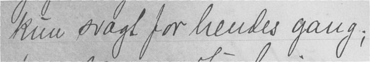kun svagt for hendes gang;
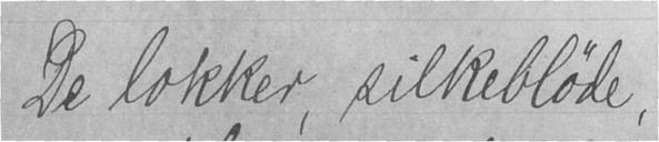De lokker, silkebløde,
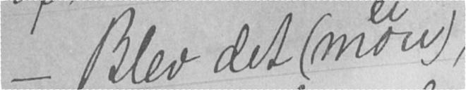- Blev det (mon)
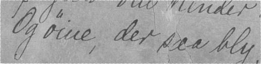Og øine, der saa bly,
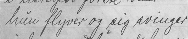hun flyver og sig svinger
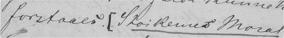forstaaes. [Stoikernes Moral
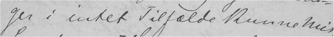ger i intet Tilfælde kunne Mis-
